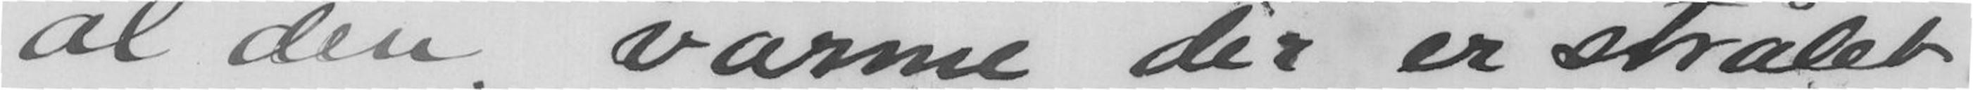al den varme der er strålet
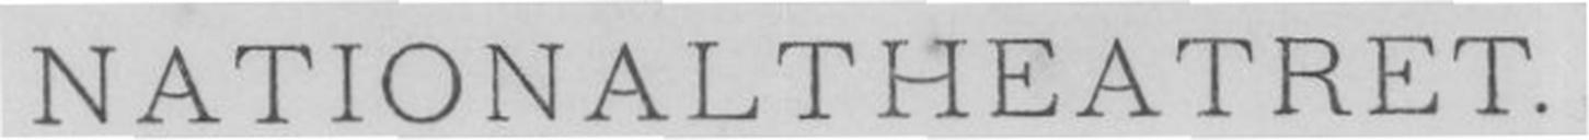NATIONALTHEATRET.
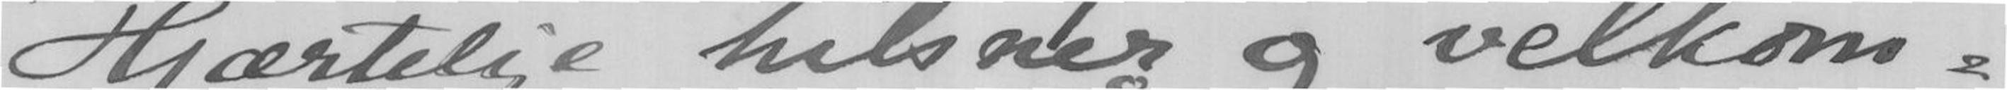Hjærtelige hilsner og velkom-
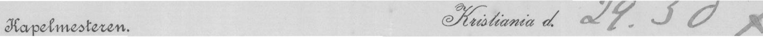Kapelmesteren.Kristiania d. 29.3 07
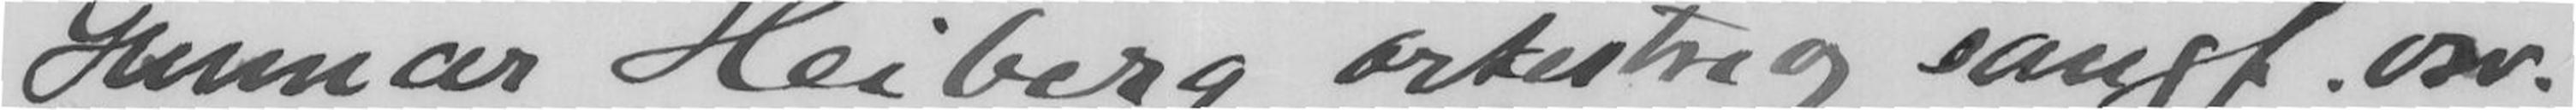Gunnar Heiberg orkestre og sangf. osv.
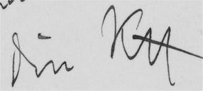Din KH
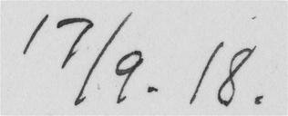17/9. 18.
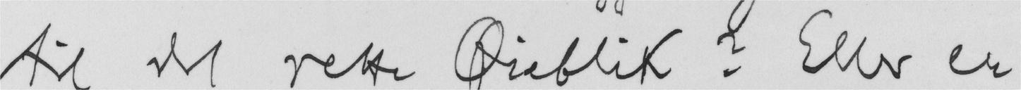til det rette Øieblik? Selv er
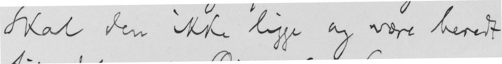Skal den ikke ligge og være beredt
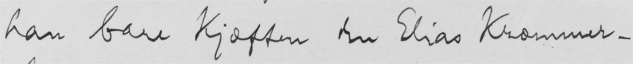han bare Kjæften den Elias Kræmmer-
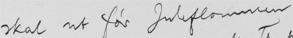skal ut før Juleflommen
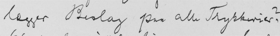lægger Beslag paa alle Trykkerier?
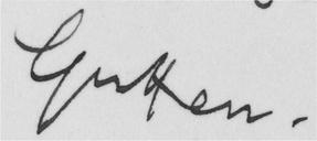Gutten.
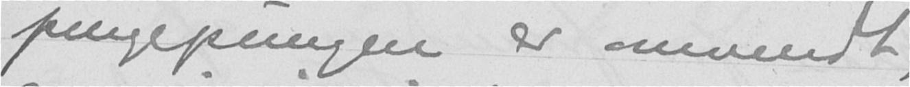pengepungen er omvendt,
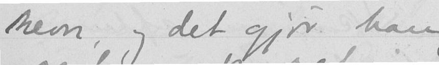hevn, og det gjør han
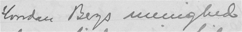Hvordan Bergs menighed
